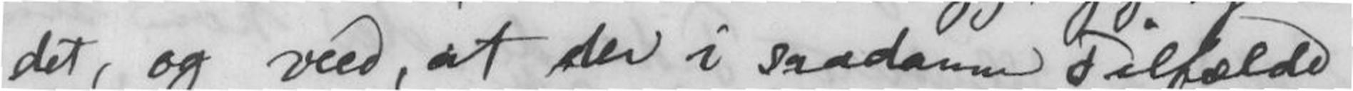det, og veed, at der i saadanne Tilfælde
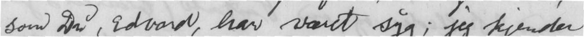som Du, Edvard, har været syg; jeg kjender
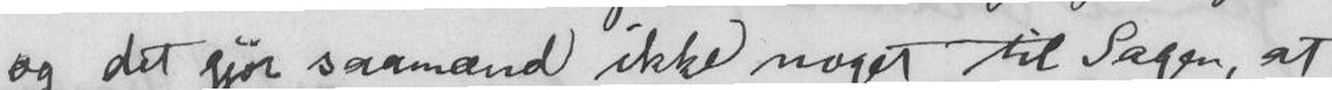og det gjør saamænd ikke noget til Sagen, at
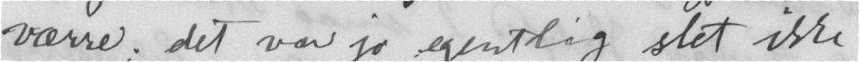værre; det var jo egentlig slet ikke
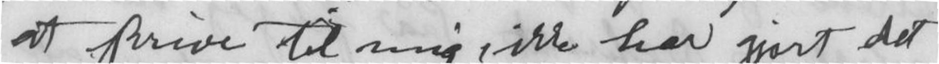at skrive til mig ikke har gjort det
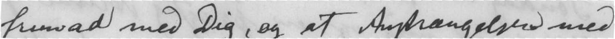fremad med Dig, og at Anstrængelsen med
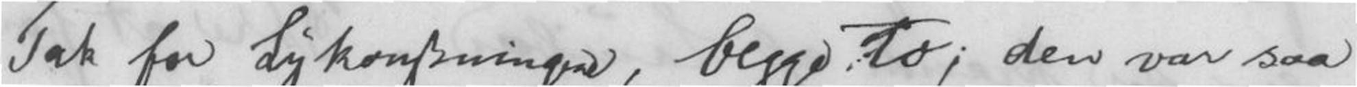Tak for Lykønskningen, begge to; den var saa
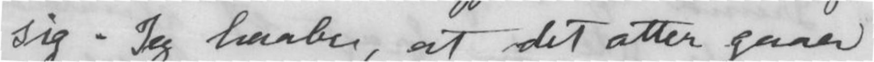sig. Jeg haaber, at det atter gaaer
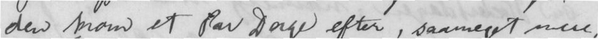den kom et Par Dage efter, saameget mere,
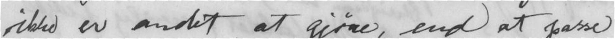ikke er andet at gjøre, end at passe
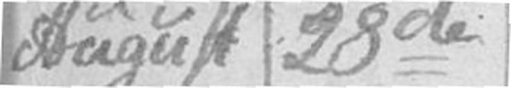August 28de
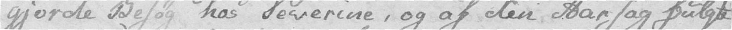gjorde Besøg hos Severine, og af den Aarsag fulgte
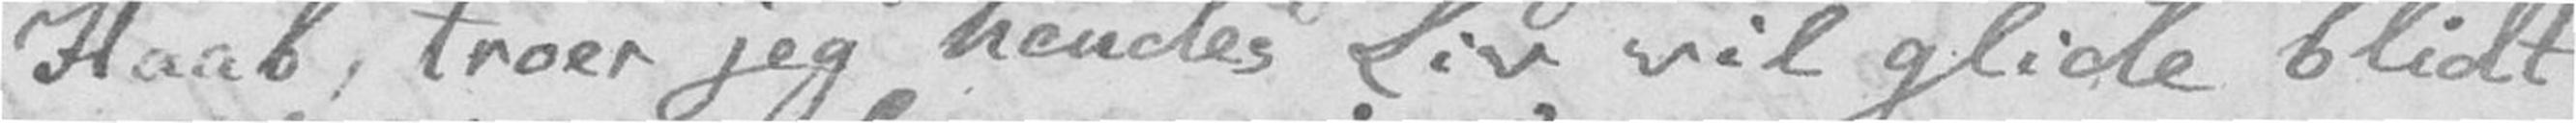Haab, troer jeg hendes Liv vil glide blidt
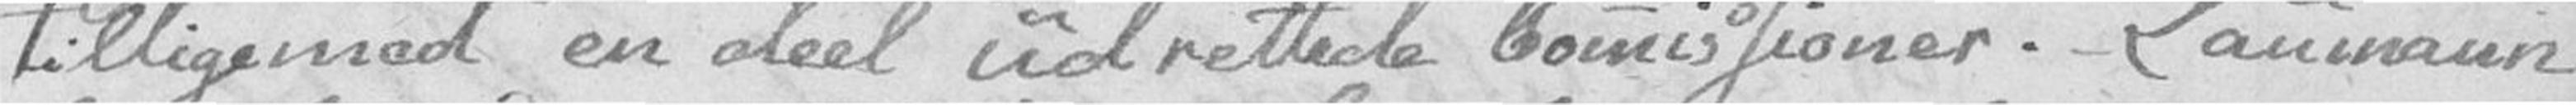tilligemed en deel udrettede Commissioner. Laumann
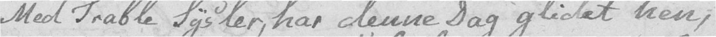Med Trable Sysler, har denne Dag glidet hen,
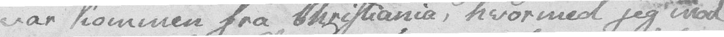var kommen fra Christiania, hvormed jeg mod
